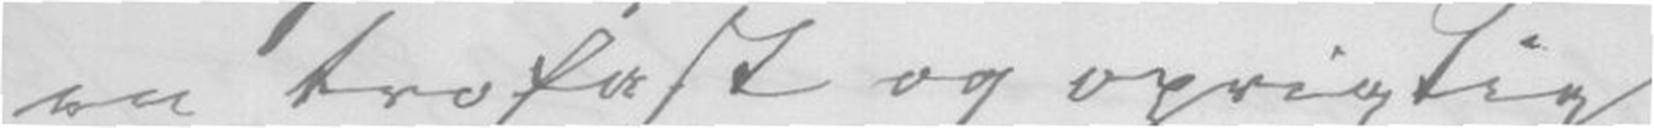en trofast og oprigtig
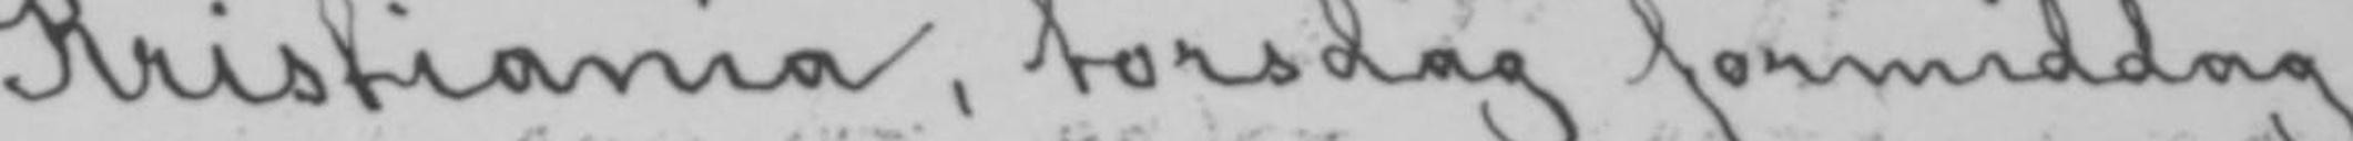Kristiania, torsdag formiddag.
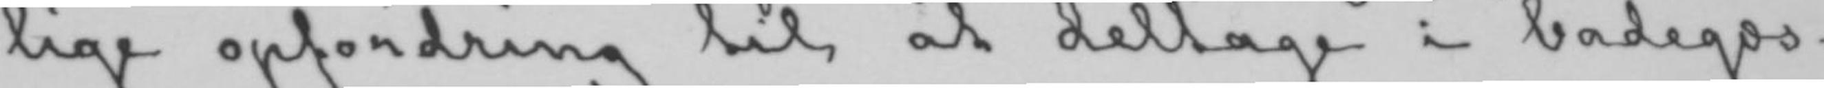lige opfordring til at deltage i badegæs-
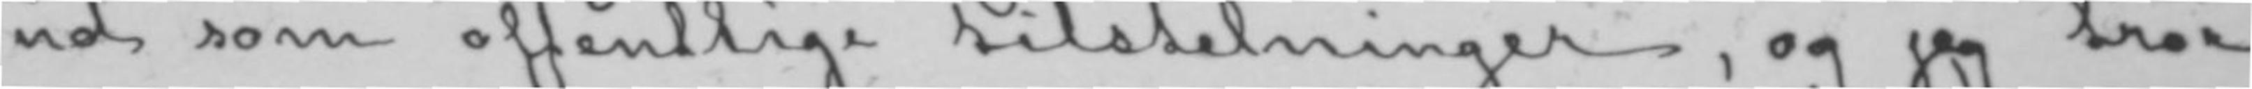ud som offentlige tilstelninger, og jeg tror
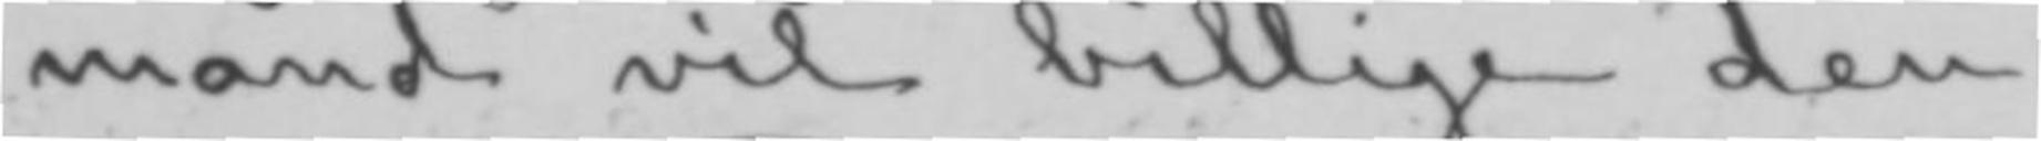mand vil billige den.
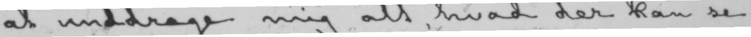at unddrage mig alt, hvad der kan se
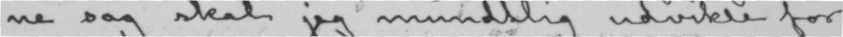ne sag skal jeg mundtlig udvikle for
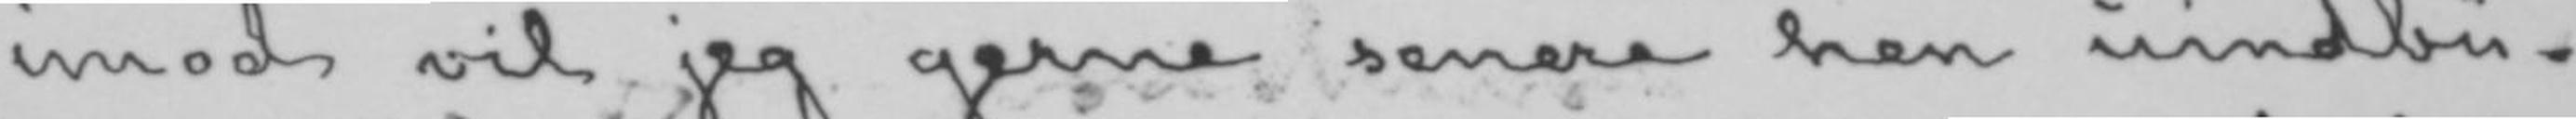imod vil jeg gerne senere hen uindbu-
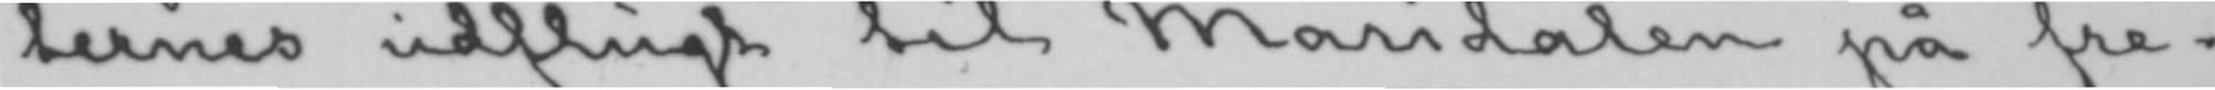ternes udflugt til Maridalen på fre-
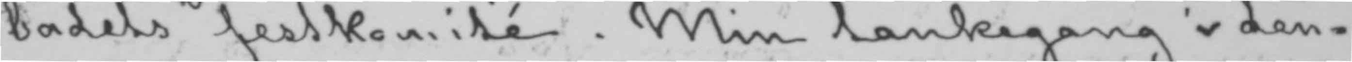badets festkomité. Min tankegang i den-
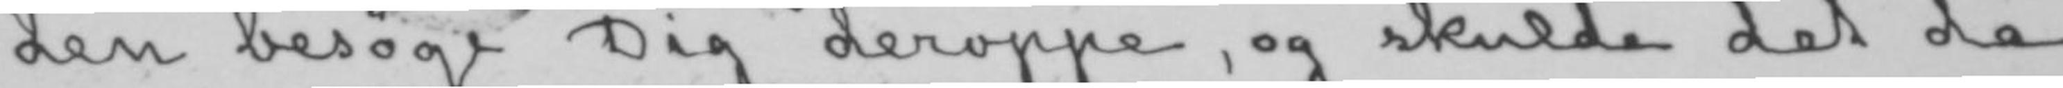den besøge Dig deroppe, og skulde det da
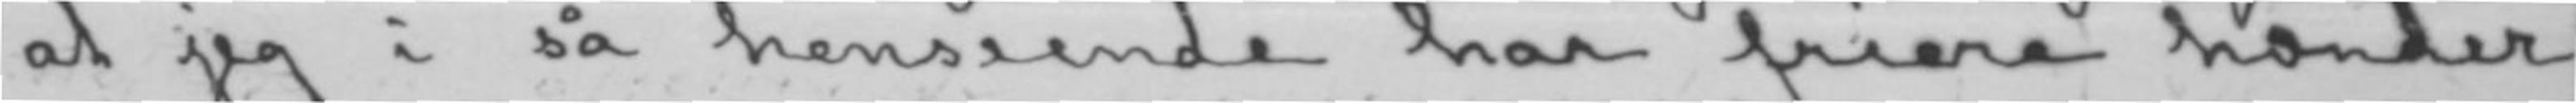at jeg i så henseende har friere hænder
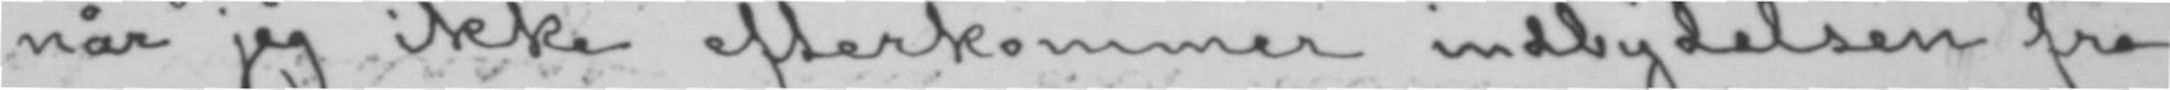når jeg ikke efterkommer indbydelsen fra
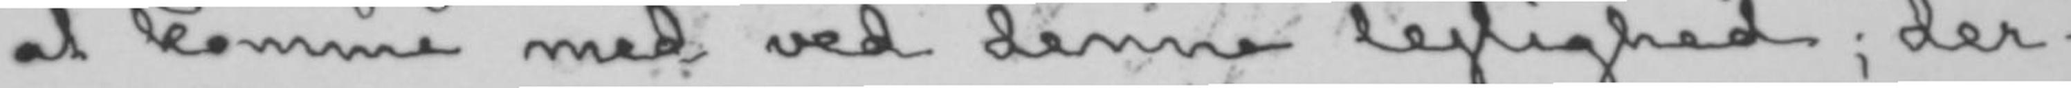at komme med ved denne lejlighed; der-
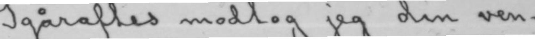Igåraftes modtog jeg din ven-
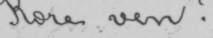Kære ven!
